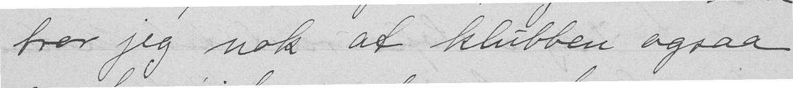tror jeg nok at klubben ogsaa
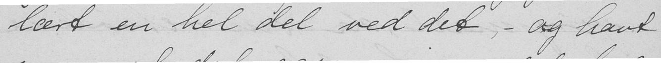lært en hel del ved det, - og havt
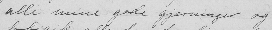alle mine gode gjerninger og
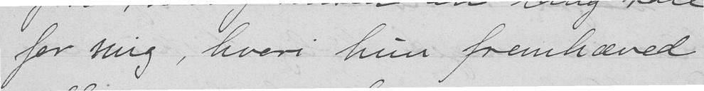for mig, hvori hun fremhæved
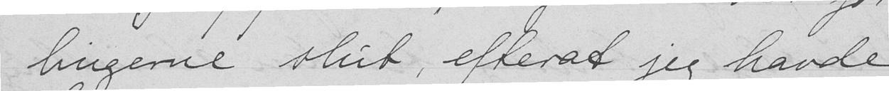lingerne slut, efterat jeg havde
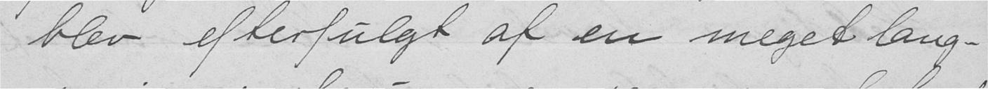blev efterfulgt af en meget lang-
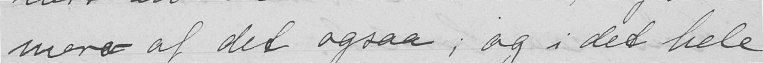moro af det ogsaa; og i det hele
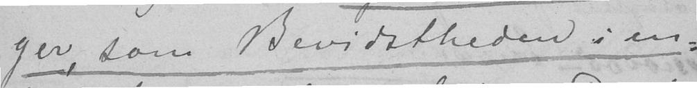ger, som Bevidstheden i en-
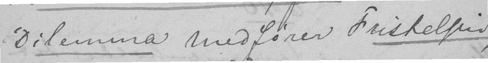Dilemma medfører Fristelsen,
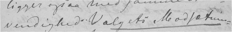vendighed Valgets Modsætnin-
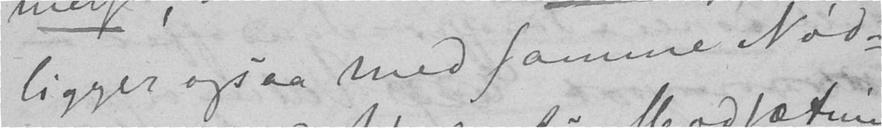ligger ogsaa med samme Nød-
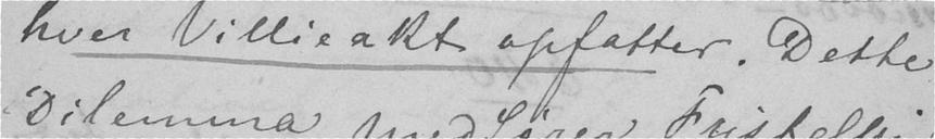hver Villiesakt opfatter. Dette
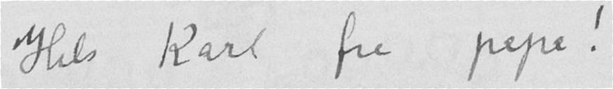Hils Karl fra pepe!
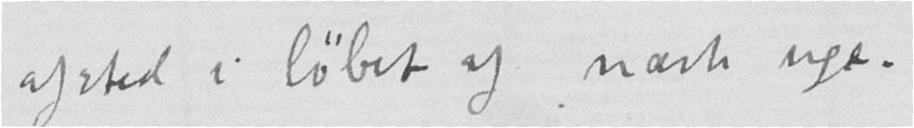afsted i løbet af næste uge.
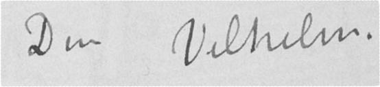Din Vilhelm.
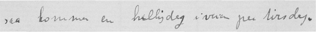saa kommer en helligdag i veien paa tirsdag.
